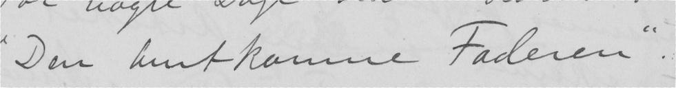"Den bortkomne Faderen".
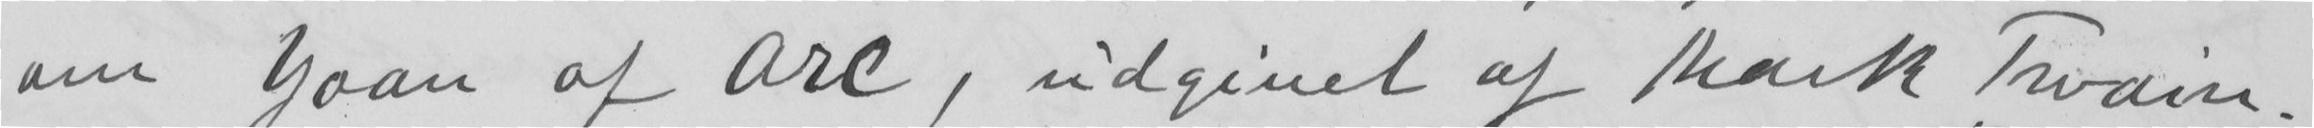om Joan of Arc, udgivet af Mark Twain.
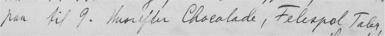paa til 9. Hvorefter Chocolade, Felespel Taler,
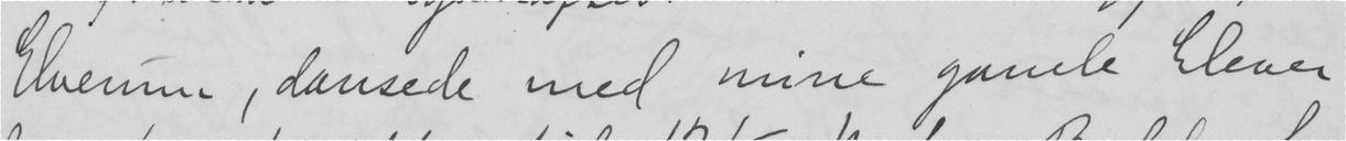Elverum, dansede med mine gamle Elever
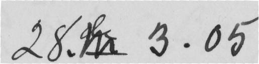28. 3.05
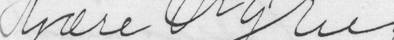Kjære Dr Grieg
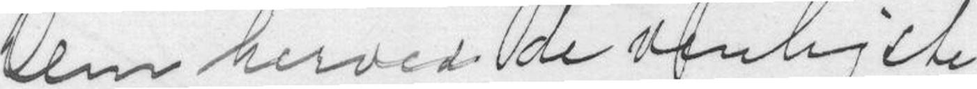Dem herved de venligste
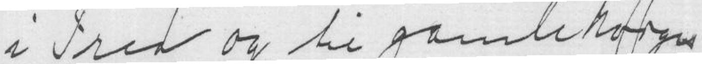i Fred og til gamle Norges
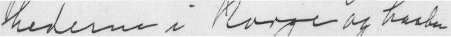hederne i Norge og haaber
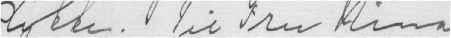Lykke. Til Fru Nina
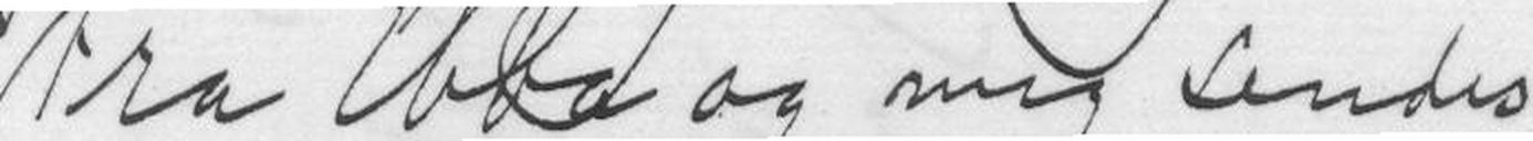Fra Ebba og mig sendes
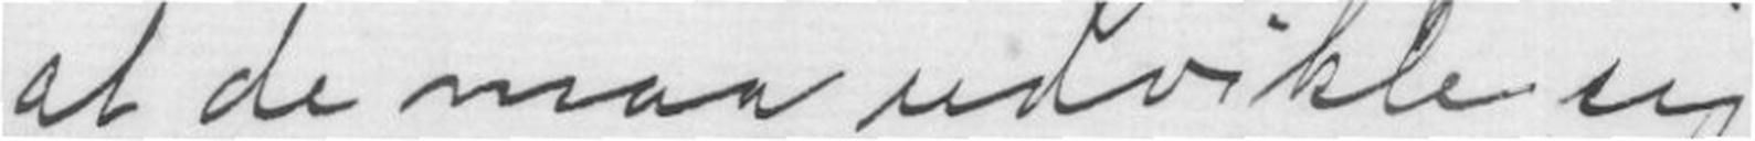at de maa udvikle sig
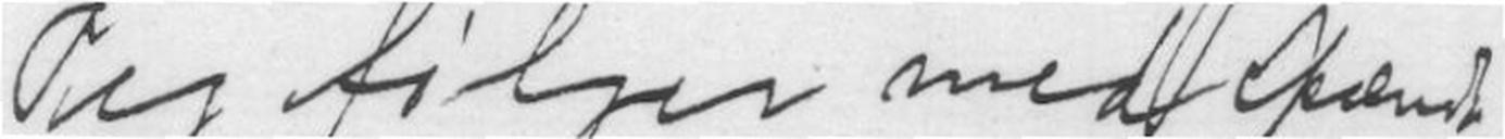Jeg følger med spændt
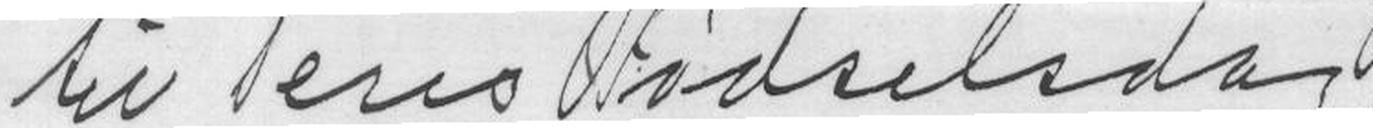til Deres Fødselsdag.
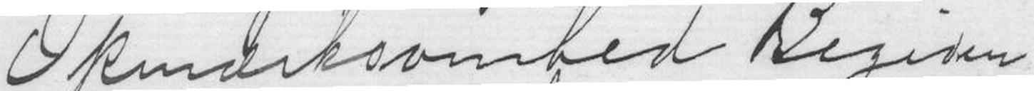Opmærksomhed Begiven-
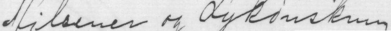Hilsner og Lykønkning
